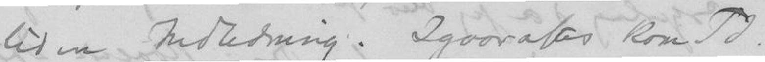liden Indledning. Igaaraftes kom Td.
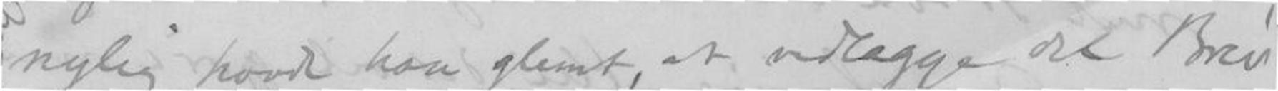nylig havde han glemt, at vedlægge det Brev
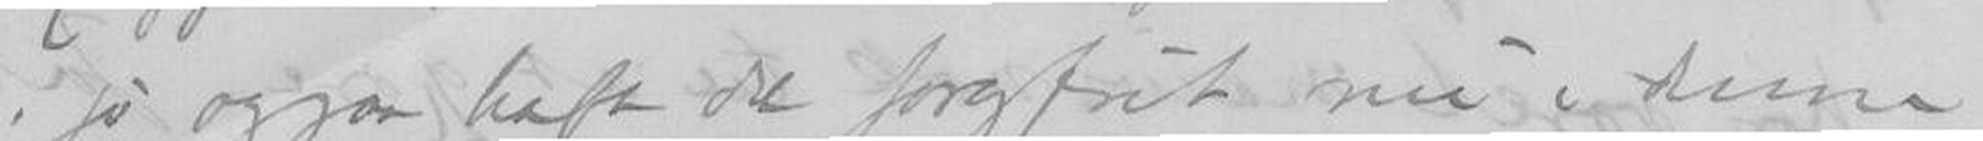jo ogsaa haft det sorgfrit nu i denne
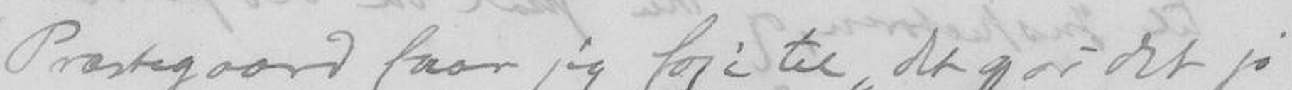Præstegaar faar jeg føje til, det gjør det jo
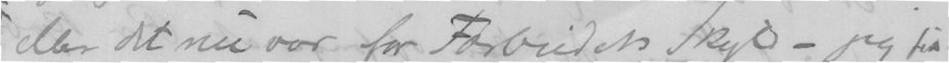eller det nu var for Forbudets skyld - jeg fik
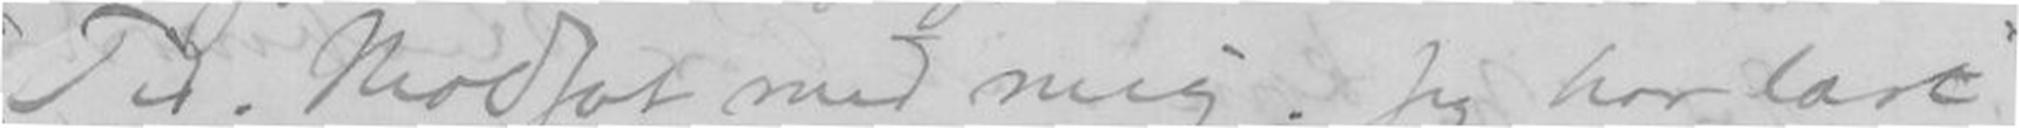Tid. Modsat med mig. Jeg har lært
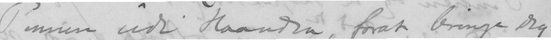Pennen udi Haanden, forat bringe Dig
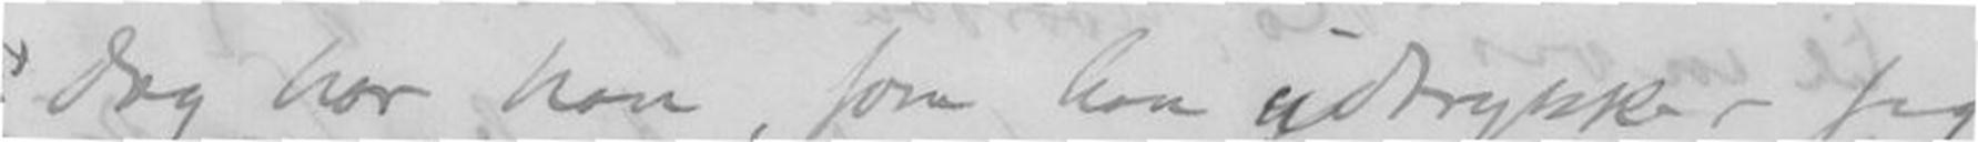dog har han, som han udtrykke sig
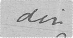din
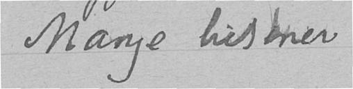Mange hilsener
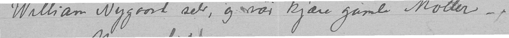William Nygaard selv, og vår kjære gamle Møller -.
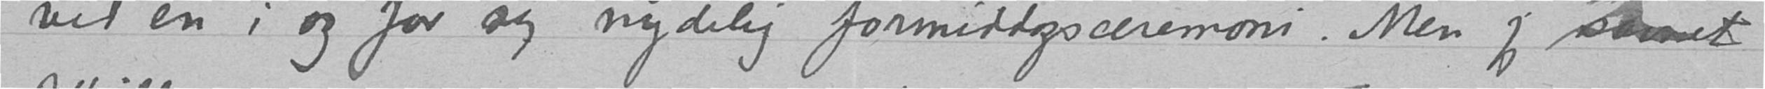ved en i og for sig nydelig formiddagsceremoni. Men jeg savnet
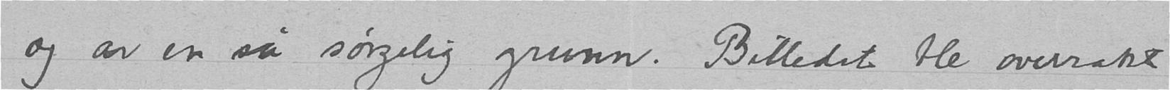og av en så sørgelig grunn. Billedet ble overrakt
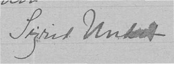Sigrid Undset
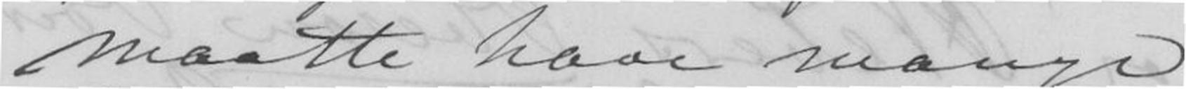maatte have mange
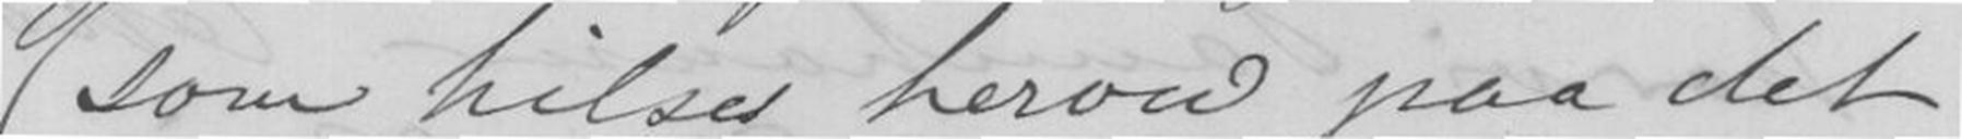(som hilses herved paa det
